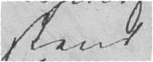stand
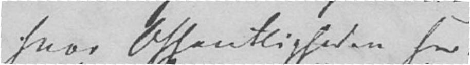hvor Offentligheden her-
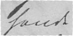havde
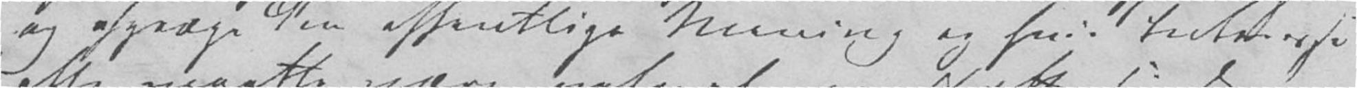og afpræge den offentlige Mening og hvis Interesse
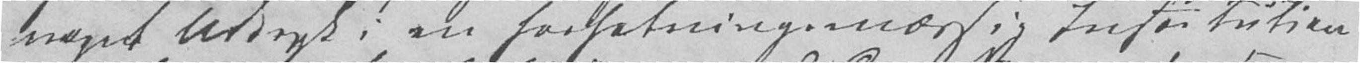noget Udtryk i en forfatningsmæssig Institution
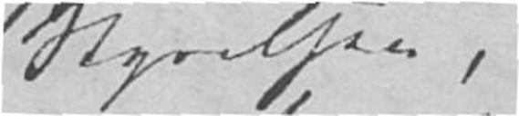Styrelsen,
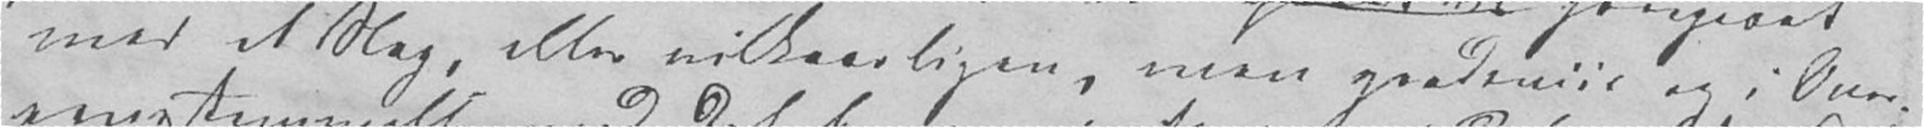med et Slag, eller vilkaarligen, men gradviis og i Over-
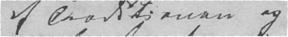af Traditionen og
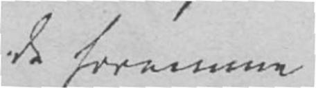de fornemme
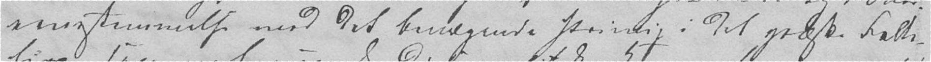ensstemmelse med det bevægende Princip i det græske Folke-
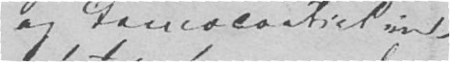og Democratiet ind-
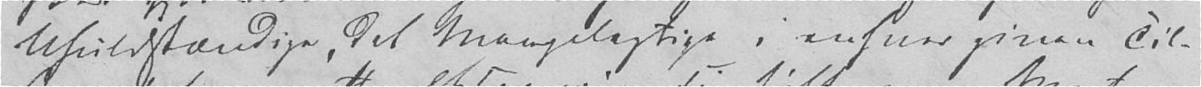Ufuldstændige, det Mangelagtige i enhver given Til-
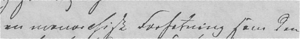en monarchisk Forfatning som den
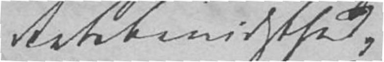Retsbevidsthed,
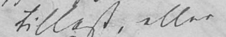tillast, eller
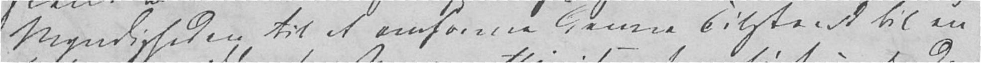Myndigheten til at omforme denne Tilstand til en
